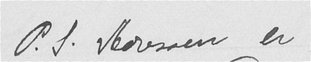P.S. Adressen er
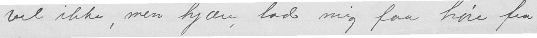vel ikke, men kjære, lad mig faa høre fra
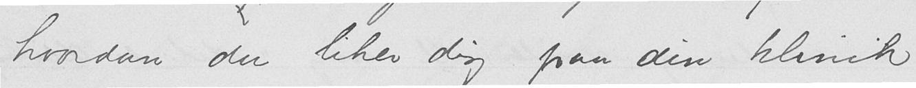hvordan du liker dig paa din klinik
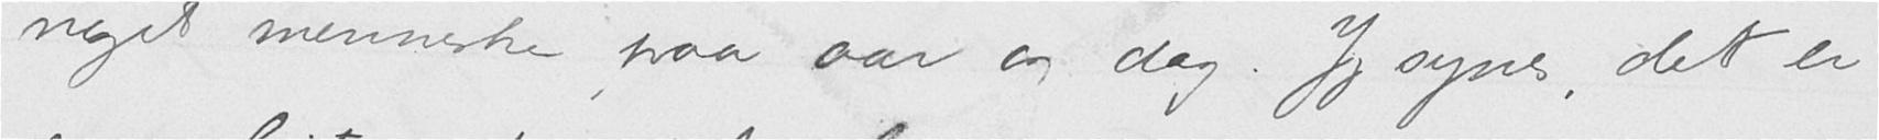noget menneske paa aar og dag. Jeg syns, det er
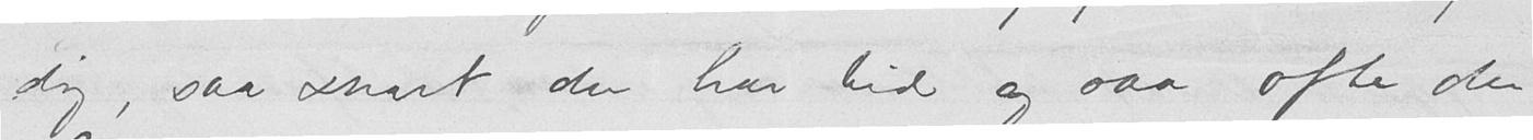dig, saa snart du har tid og saa ofte du
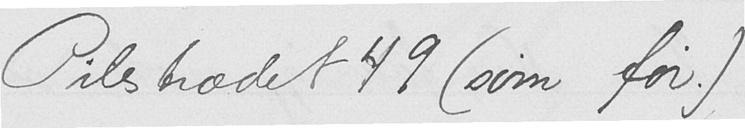Pilestrædet 49 (som før.)
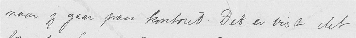naar jeg gaar paa kontoret. Det er vist det
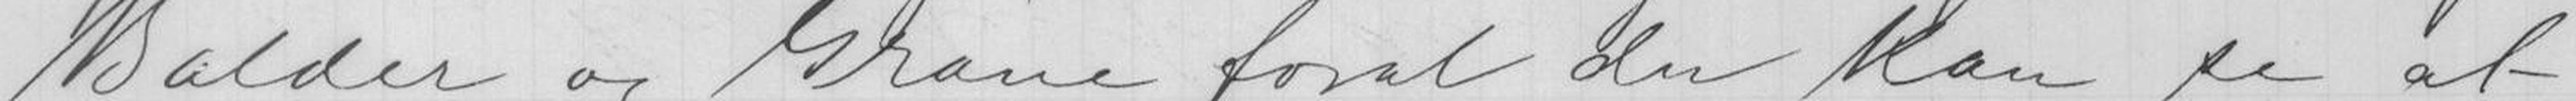Balder og Grane forat du kan se at
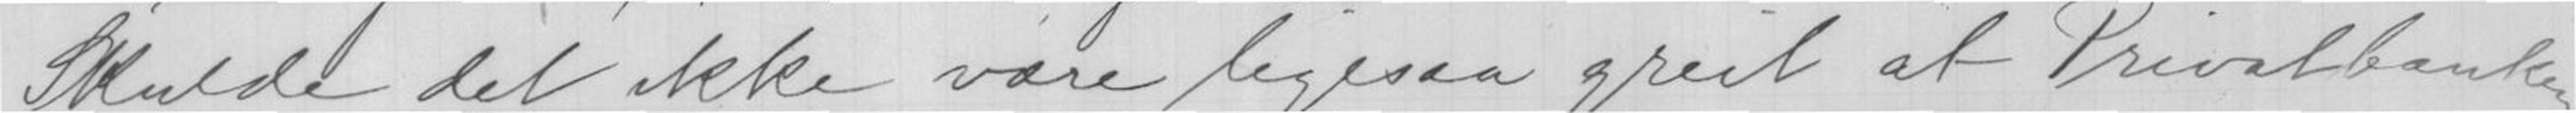Skulde det ikke være ligesaa greit at Privatbanken
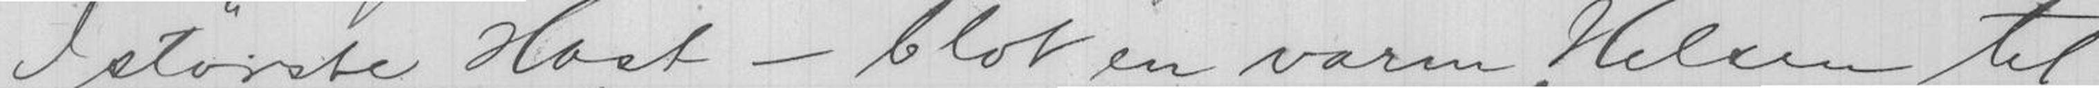I største Hast - blot en varm Hilsen til
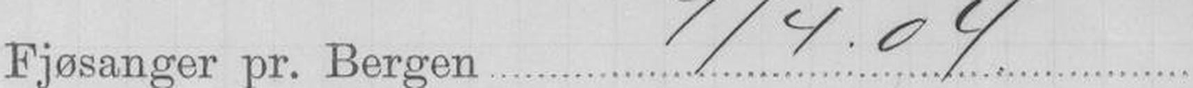Fjøsanger pr. Bergen 9/4.04
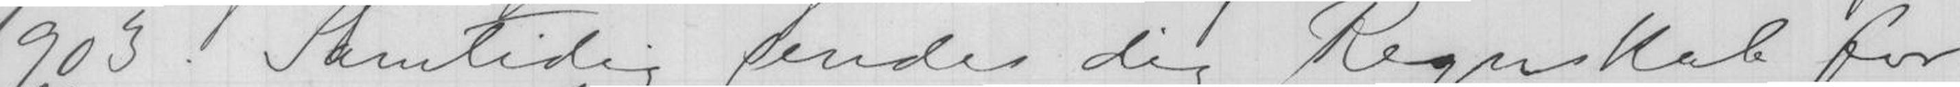1903. Samtidig sendes dig Regnskab for
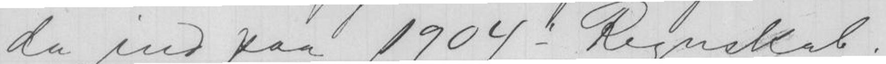da ind paa 1904s Regnskab.
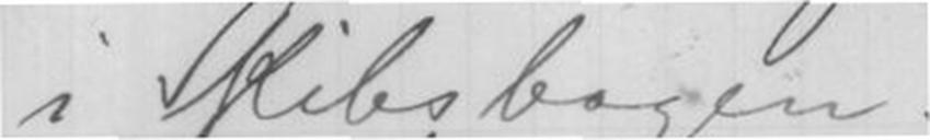i Skibsbogen.
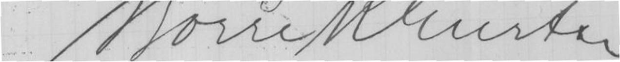Børre R Giertsen
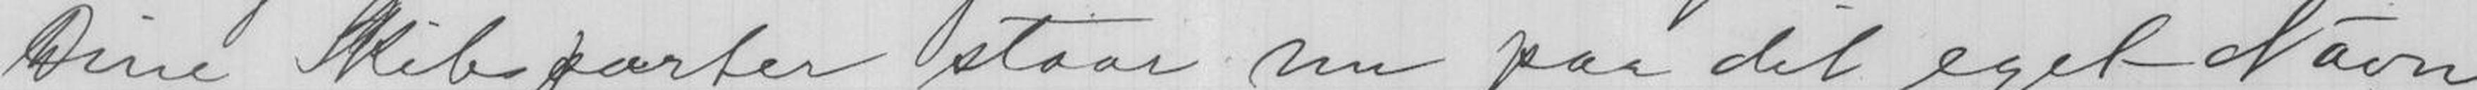Dine Skibsparter staar nu paa dit eget Navn
